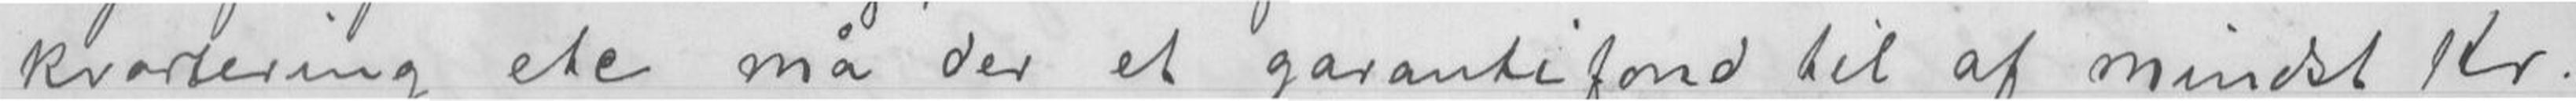kvatering etc. må der et garantifond til af mindst Kr.
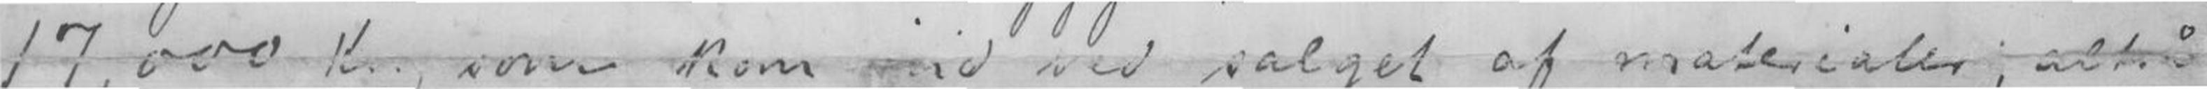- 17.000 Kr.; som kom ind ved salget af materiale; altså
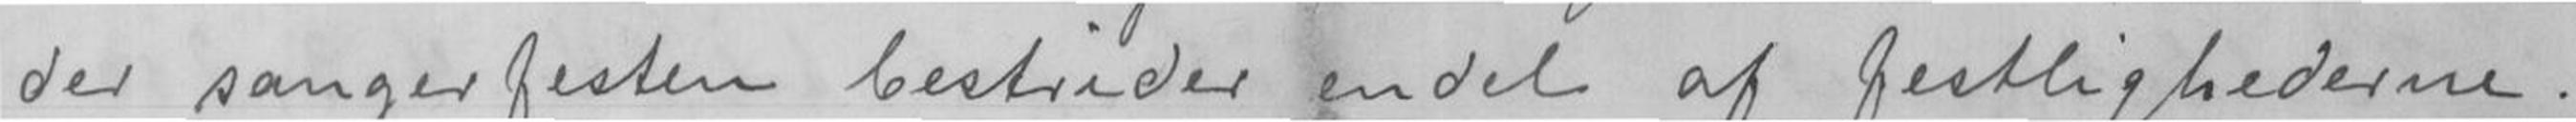der sangerfesten bestrider endel af festlighederne.
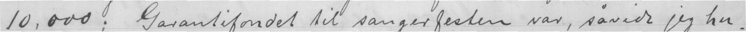10.000; Garantifondet til sangerfesten var, såvidt jeg hu-
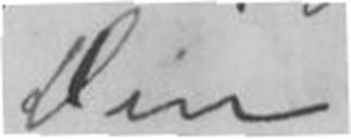Din
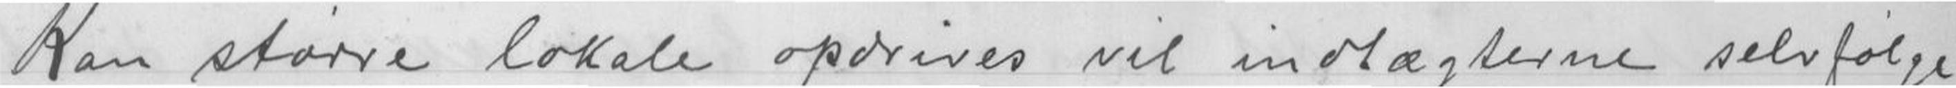Kan større lokale opdrives vil indlægterne selvfølge-
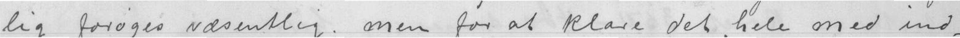lig forøges væsentlig; men for at klare det hele med ind-
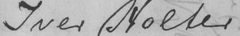Iver Holter.
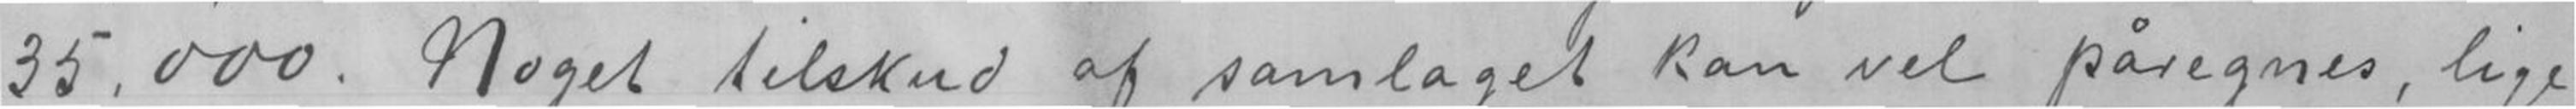35.000 Noget tilskud af samlaget kan vel påregnes, lige
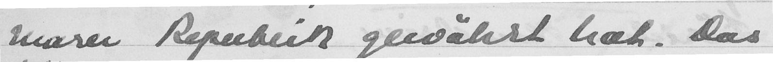marer Pepeblik gesøkst nat. Dos
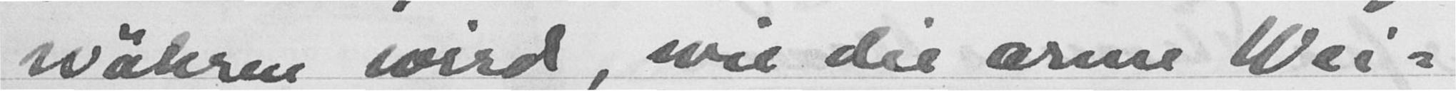ahren mird, svee du arme Wei-
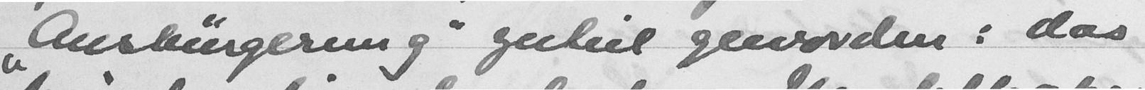"Ansbugering" guteil gevørden i das
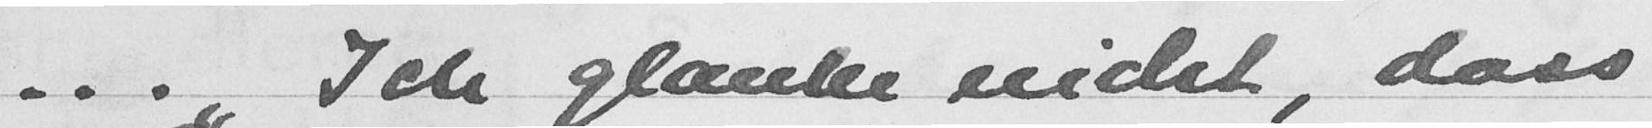-.. Sch glanke endet, dass
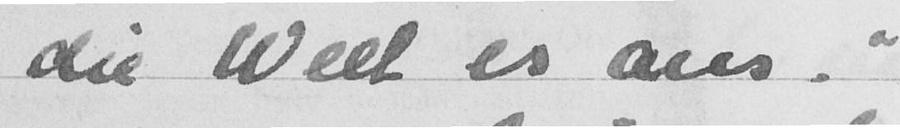du Weet es ans."
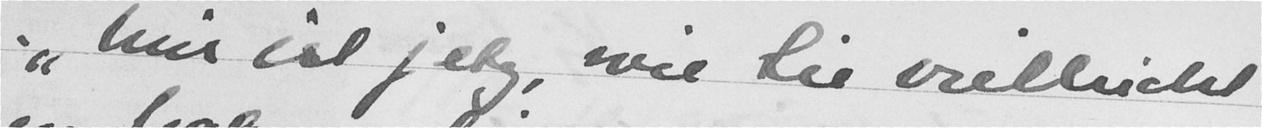ner est jehg, veie tie vcelliicht
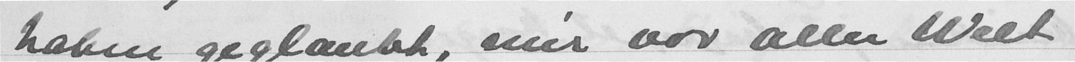haven geglaubt, nur var alen dcet
